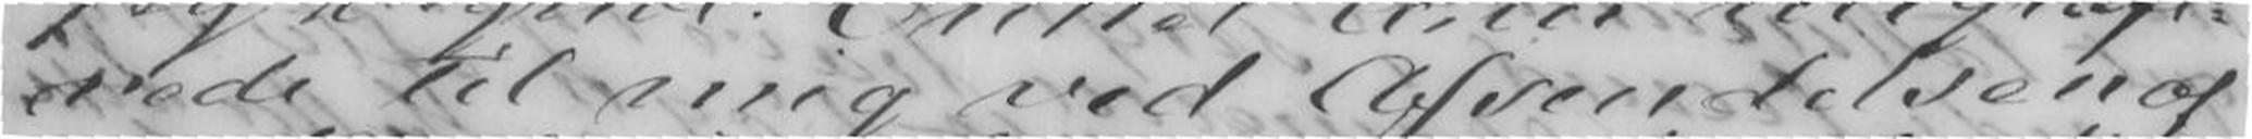rede til mig ved Afsendelsen af
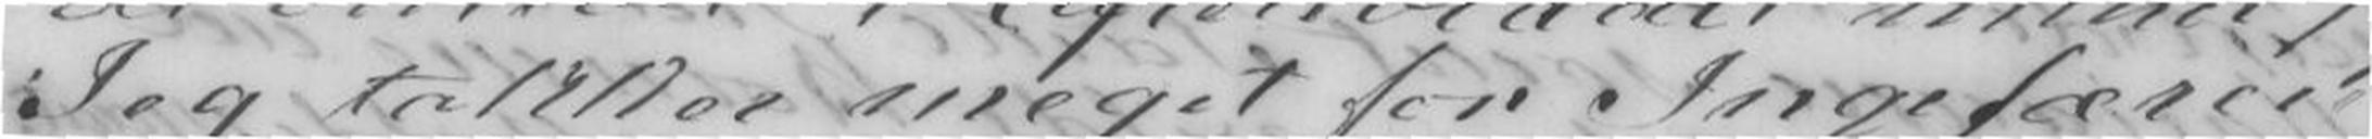Jeg takker meget for Ingefærer,
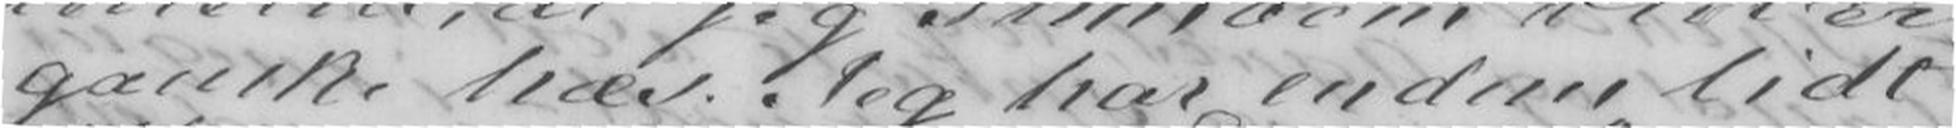ganske Hæs. Jeg har endnu lidt
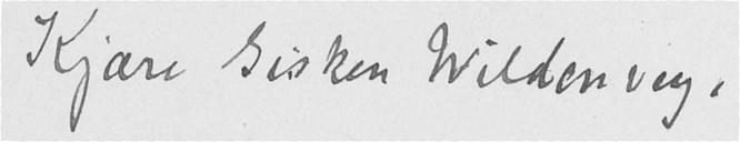Kjære Gisken Wildenvey,
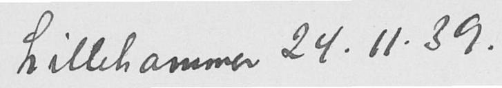Lillehammer 24.11.39.
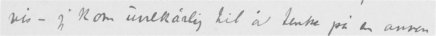vis – jeg kom uvilkårlig til å tenke pa en annen
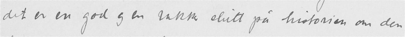det er en god og en vakker slutt paa historien om den
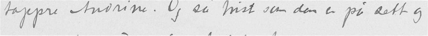tappre Andrine. Og så trist som den er på sett og
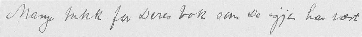Mange takk for Deres bok som De igjen har vært
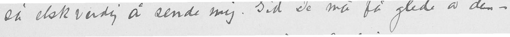så elskverdig å sende mig. Gid De må få glede av den –
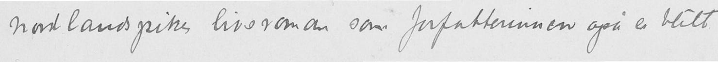nordlandspikes livsroman som forfatterinnen også er blitt
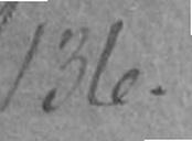136.
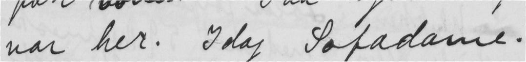var her. Idag Sofadame! madraase
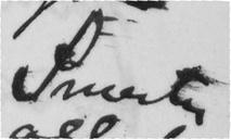Smerte
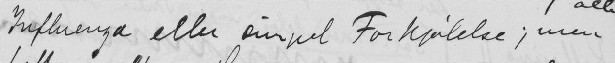Influenza eller singel Forkjølelse; men
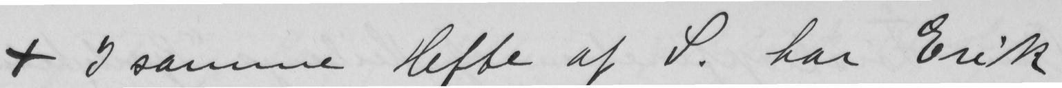x I samme Hefte af S. har Erik
